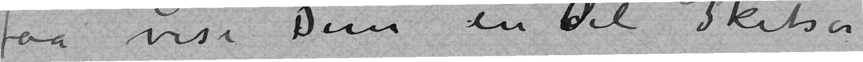faa vise Dem en Del Skitser
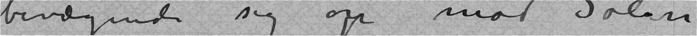bevægende sig op mod Solen
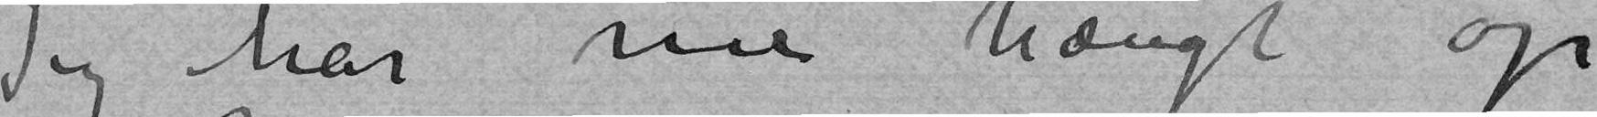Jeg har nu hængt op
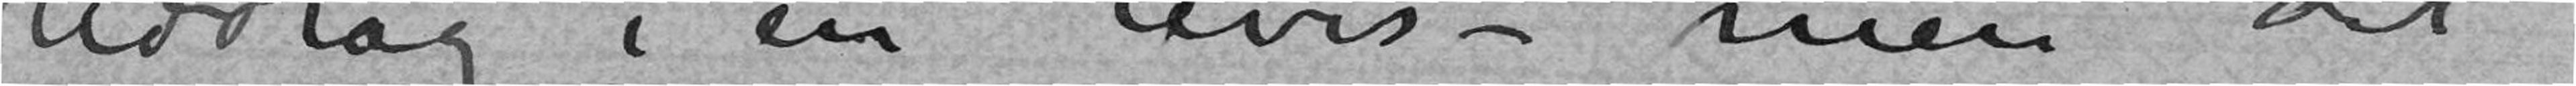Uddrag i en Avis – men det
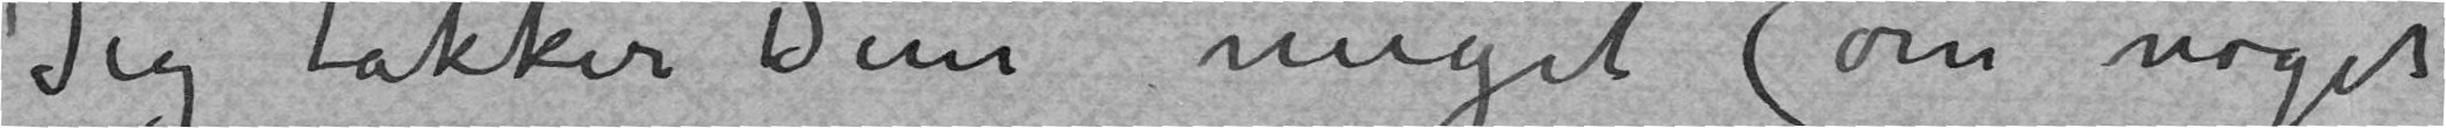Jeg takker Dem meget (om noget
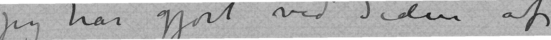jeg har gjort ved Siden af
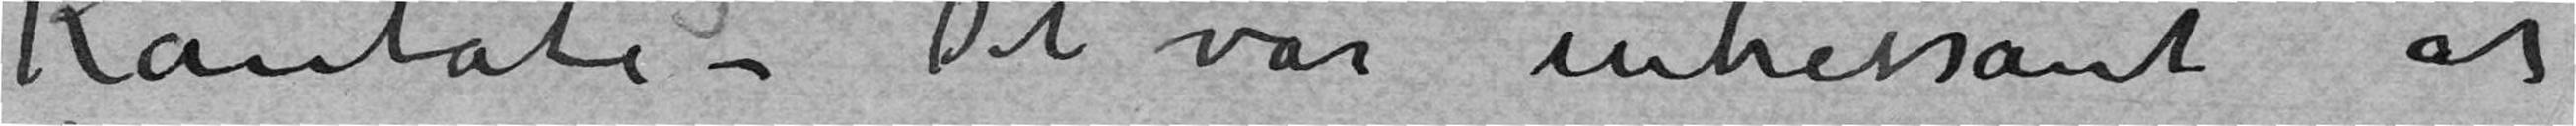Kantate – De var intressant at
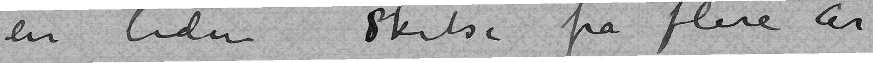en liden Skitse fra flere År
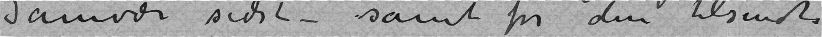Samvær sidst – samt for den tilsendte
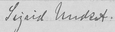Sigrid Undset.
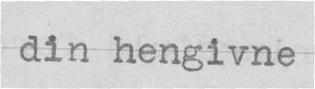din hengivne
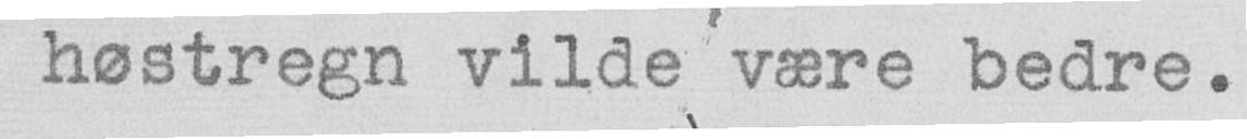høstregn vilde være bedre.
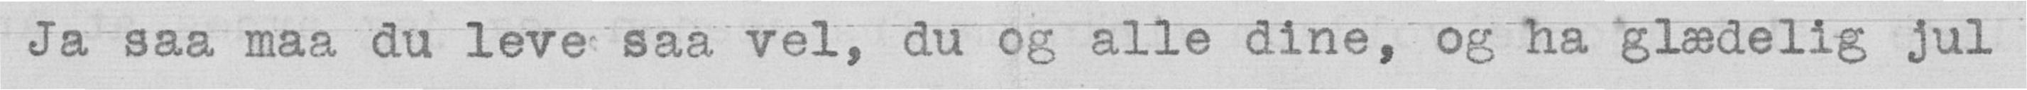Ja saa maa du leve saa vel, du og alle dine, og ha glædelig jul
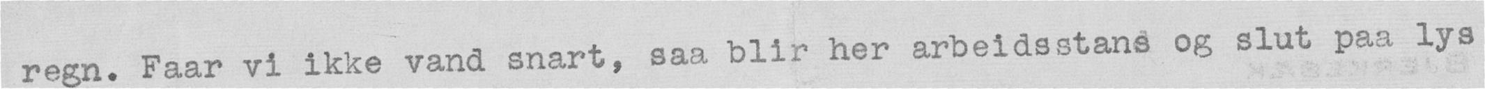regn. Faar vi ikke vand snart, saa blir her arbeidsstans og slut paa lys
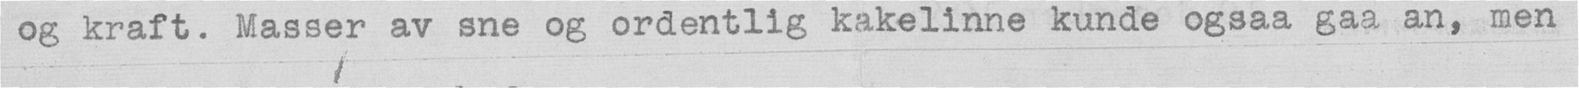og kraft. Masser av sne og ordentlig kakelinne kunde ogsaa gaa an, men
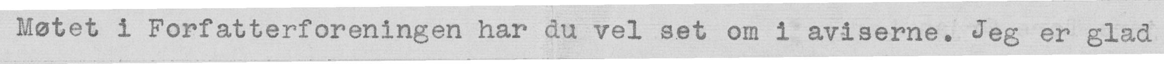Møtet i Forfatterforeningen har du vel set om i aviserne. Jeg er glad
